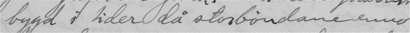bygd i tider då storböndane enno
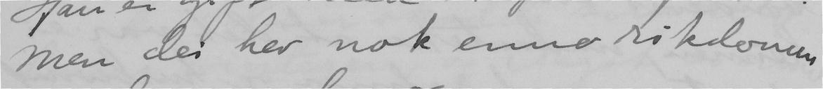Men dei hev nok enno rikdomen
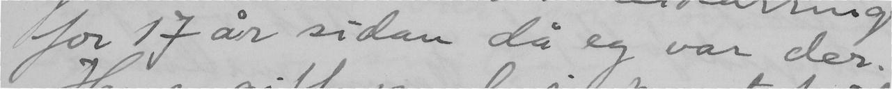for 17 år sidan då eg var der.
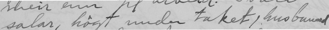salar, högt under taket, husbunad
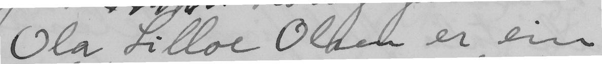Ola Lilloe Olsen er ein
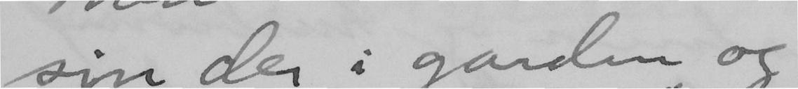sin dei i garden og.
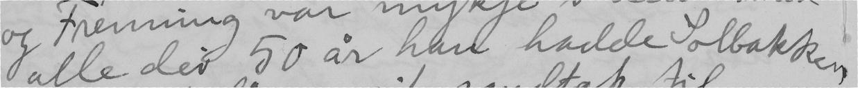alle dei 50 år han hadde Solbakken.
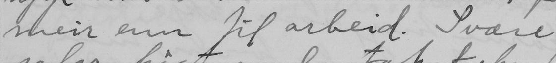meir enn til arbeid. Svære
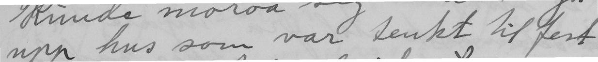upp hus som var tenkt til fest
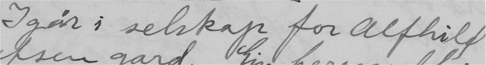Igår i selskap for Alfhild
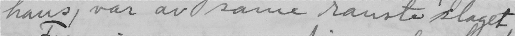hans, var av same rauste slaget,
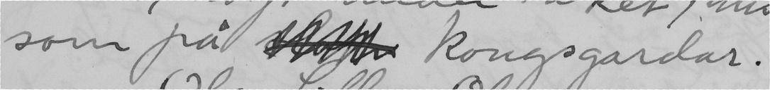som på  kongsgardar.
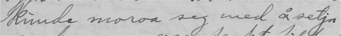kunde moroa sig med å setja
